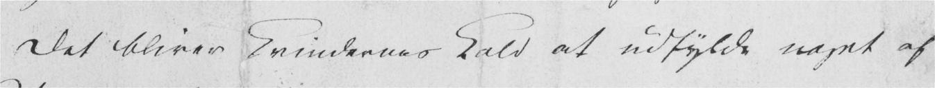Det bliver Kvindernes Kald at udfylde noget af
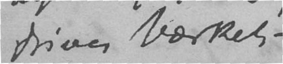driver Værket"
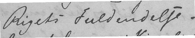Rigets Fuldendelse.
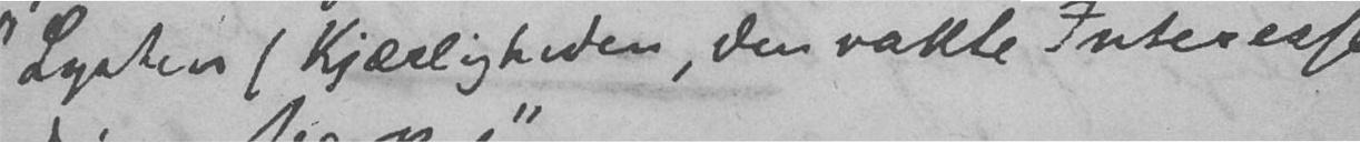"Lysten (Kjærligheden, den vakte Interesse),
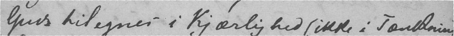Gud tilegnes i Kjærlighed (ikke i Tænkning)
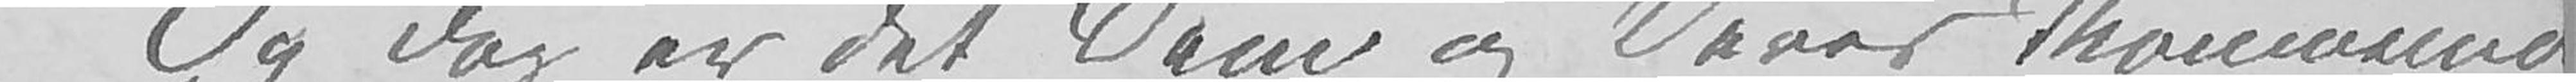Og dog er det Dem og Deres Thomasine
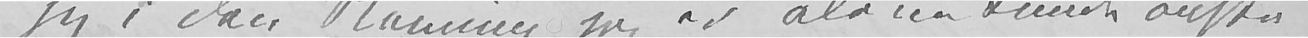jeg i den Stemning jeg er alene kunde ønske
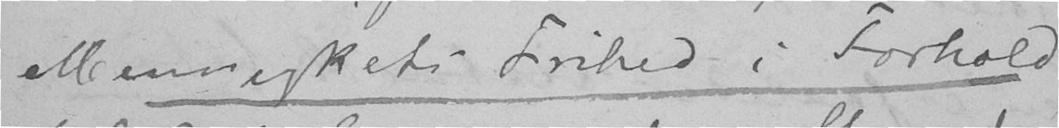Menneskets Frihed i Forhold
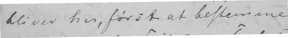bliver her, først at bestemme
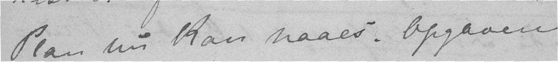Plan nu kan naaes. Opgaven
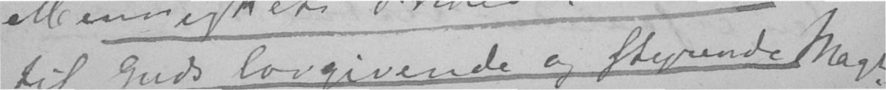til Guds lovgivende og styrende Magt
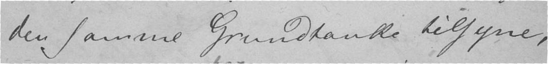den samme Grundtanke tilsyne,
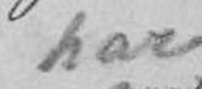har
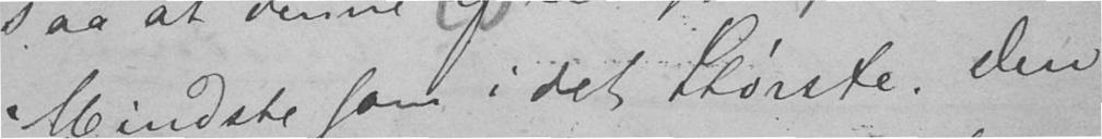Mindste som i det Største. Den
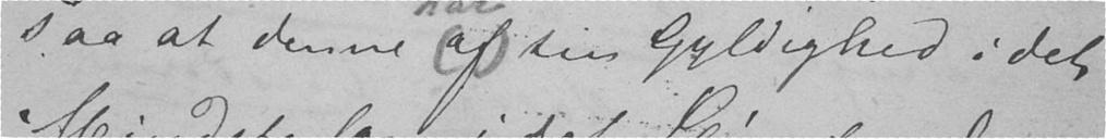saa at denne af sin Gyldighed i det
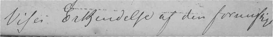Vises Erkjendelse af den fornuftige
